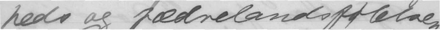heds og fædrelandsfølelsen
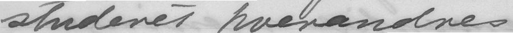studeret hverandres
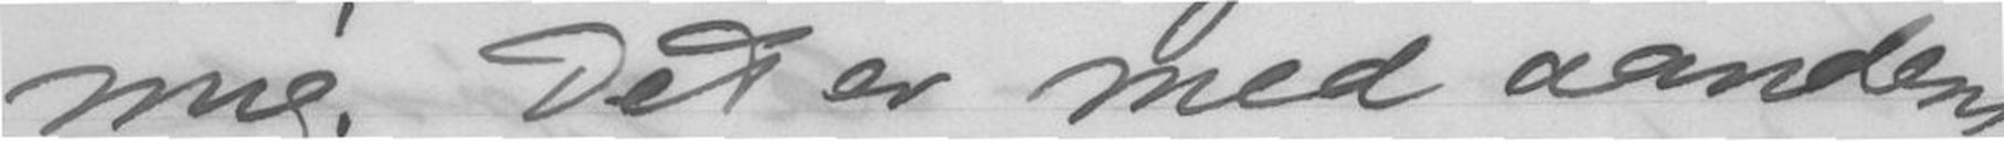mig. Det er med aandens
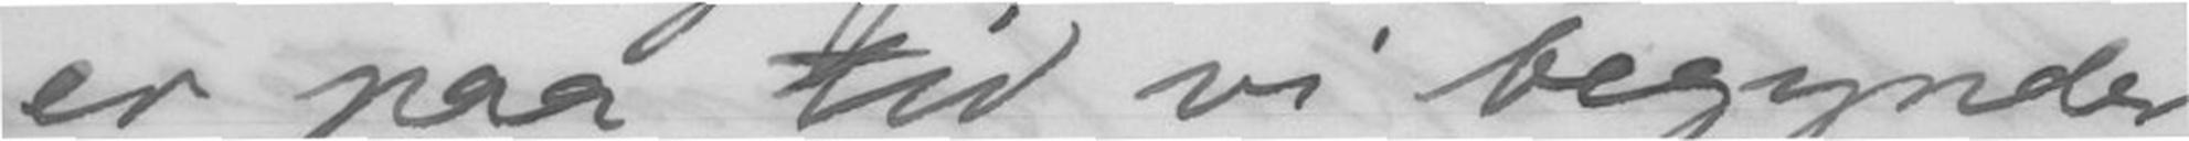er paa tid vi begynder
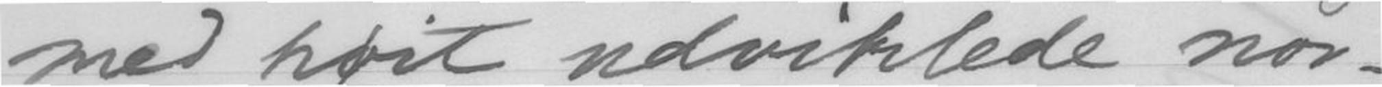med høit udviklede nor-
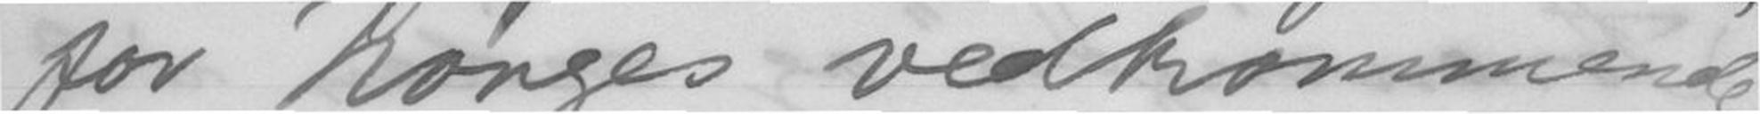for Norges vedkommende
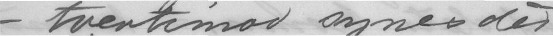- tvertimod synes det
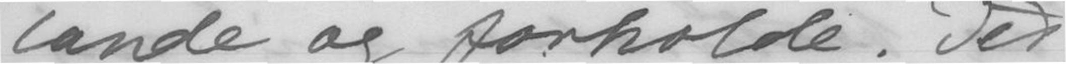lande og forholde. Det
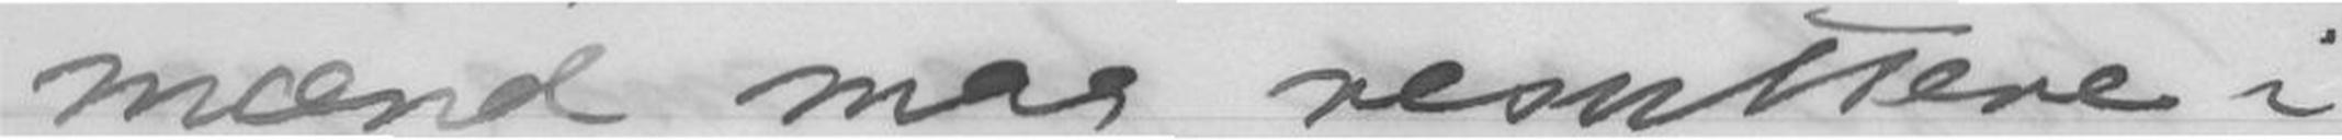mænd maa resultere i
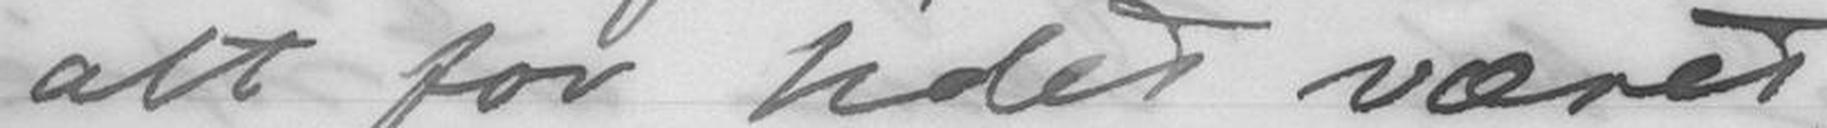alt for lidet været
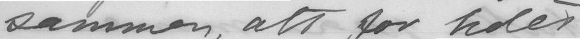sammen, alt for lidet
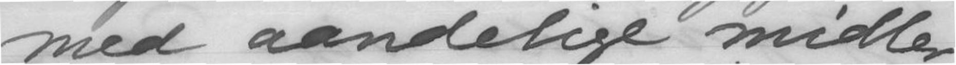med aandelige midler.
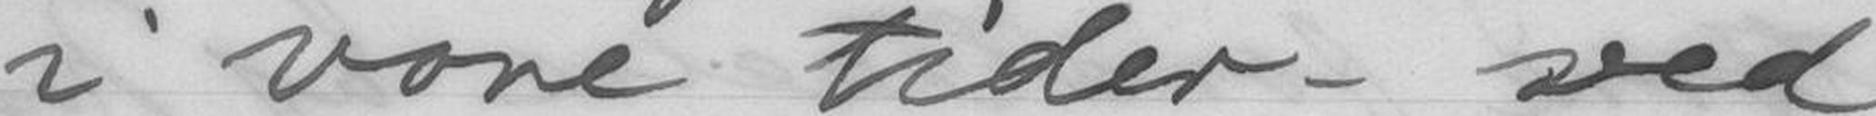i vore tider - ved
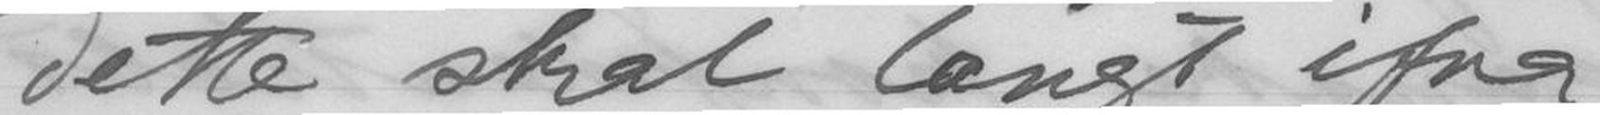Dette skal langt ifra
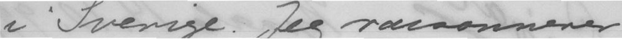i Sverige Jeg raisonnerer
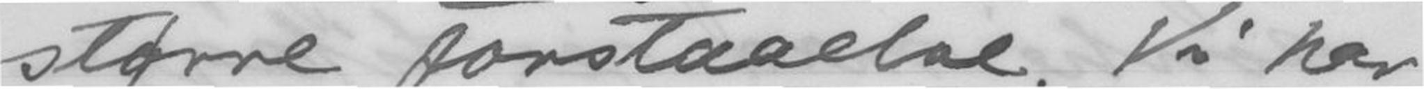større forstaaelse. Vi har
